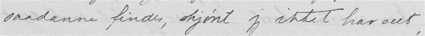saadanne findes, skjønt jeg intet har seet,
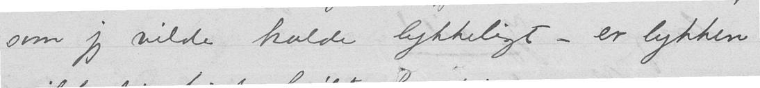som jeg vilde kalde lykkeligt - er lykken
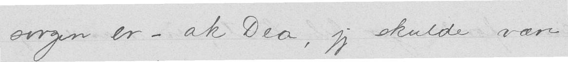sorgen er - ak Dea, jeg skulde være
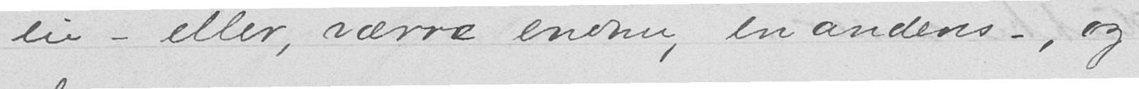eie - eller, værre endnu, en andens -, og
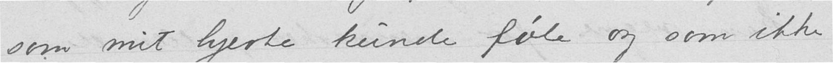som mit hjerte kunde føle og som ikke
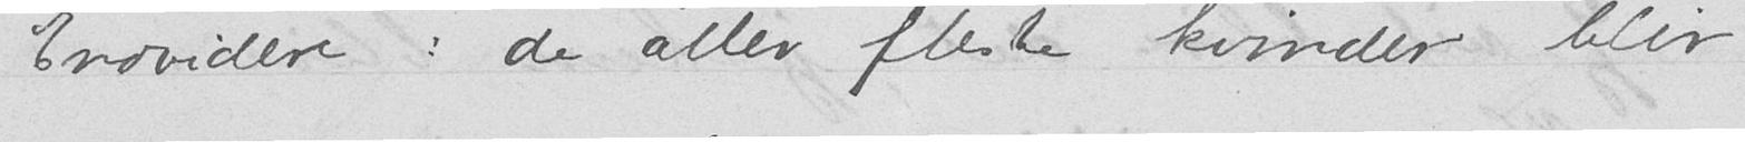Endvidere: de aller fleste kvinder blir
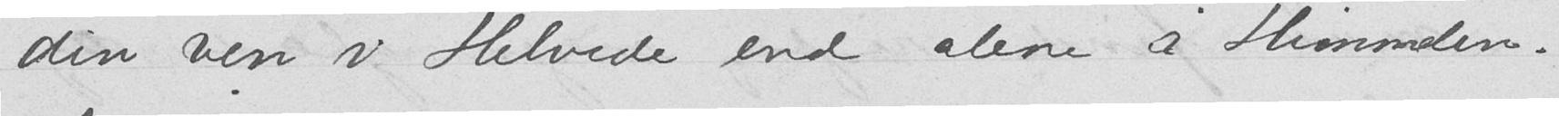din ven i Helvede end alene i Himmelen.
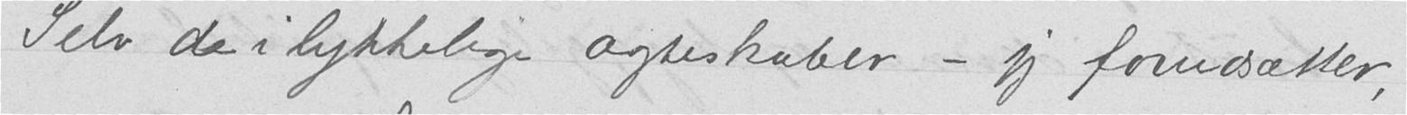Selv de i lykkelige ægteskaber - jeg forudsætter,
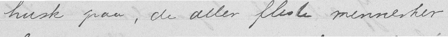husk paa, de aller fleste mennesker
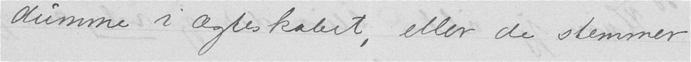dumme i ægteskabet, eller de stemmer
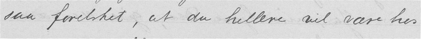saa forelsket, at du hellere vil være hos
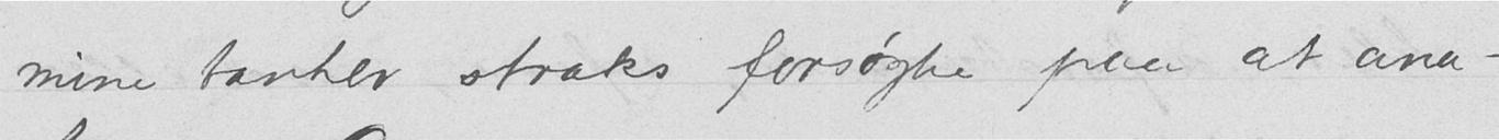mine tanker straks forsøgte paa at ana-
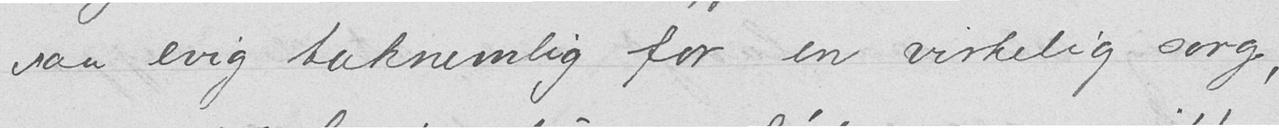saa evig taknemlig for en virkelig sorg,
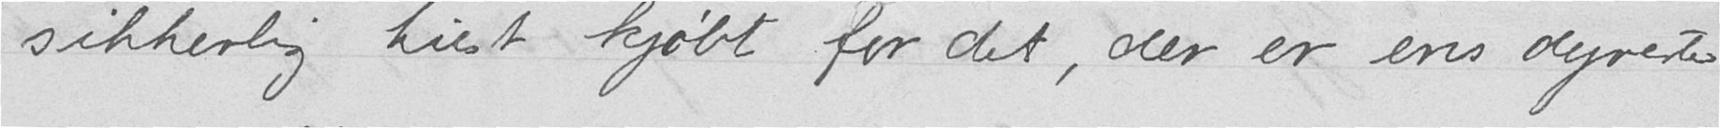sikkerlig helst kjøbt for det, der er ens dyreste
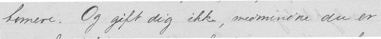tomere. Og gift dig ikke, medmindre du er
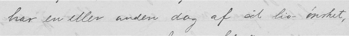har en eller anden dag af sit liv ønsket,
